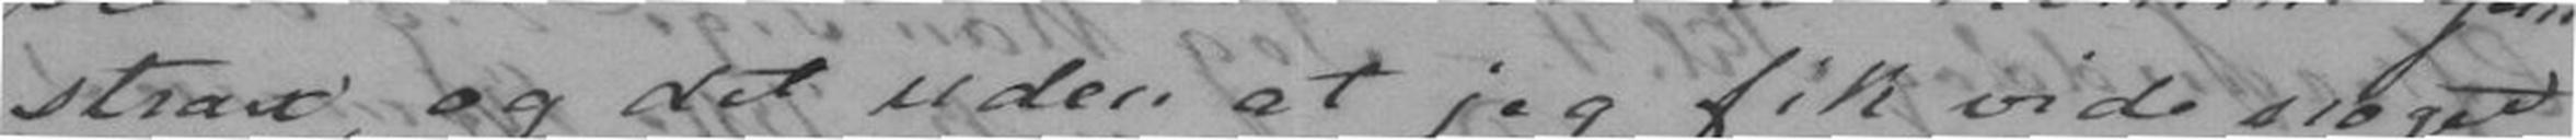strax, og det uden at jeg fik vide noget
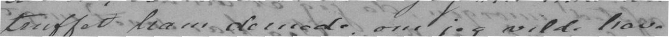truffet ham dernede, om jeg vilde have
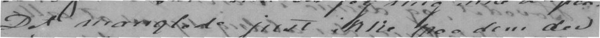Det manglede just ikke paa dem der
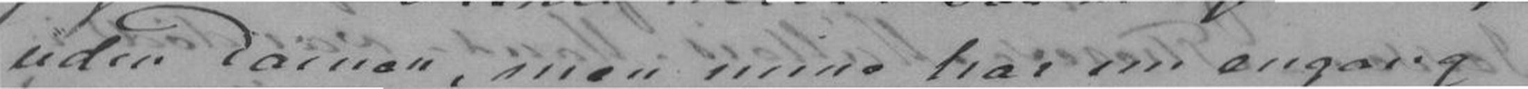uden Damen, men mine har nu engang
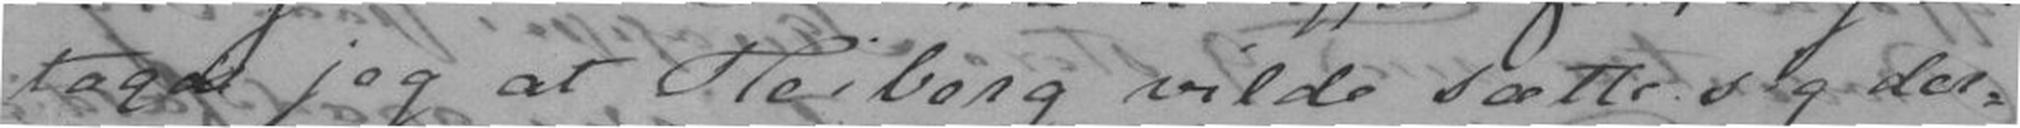tager jeg at Heiberg vilde sætte sig der-
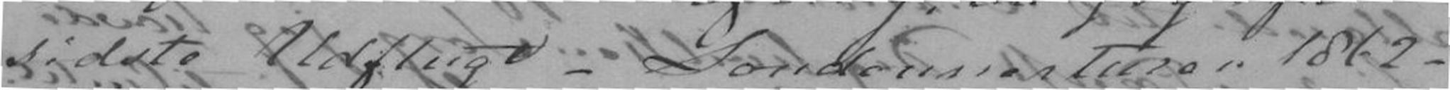sidste udflugt - Londonnerturen i 1862 -
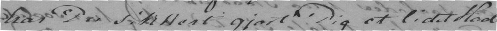har Du sikkert gjort Dig et lidet Haab
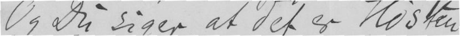Og Du siger at det er Høsten,
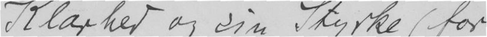Klarhed og sin Styrke (for
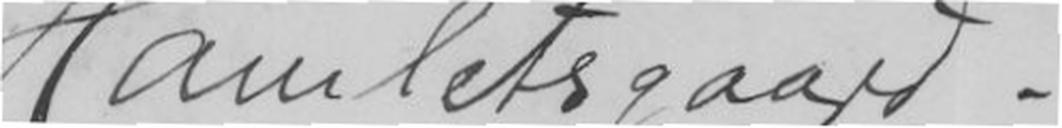Hamletsgaard.
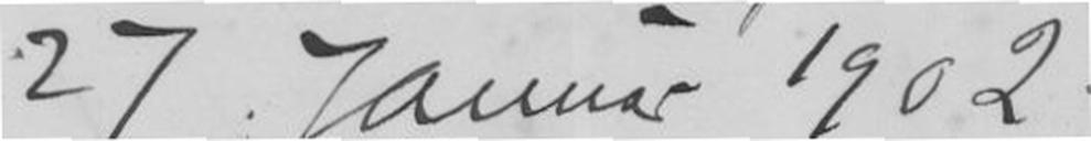27 Januar 1902.
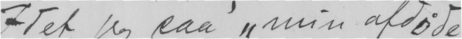I det jeg saa min afdøde
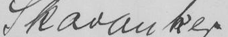Skavanker.
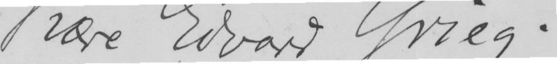Kære Edvard Grieg.
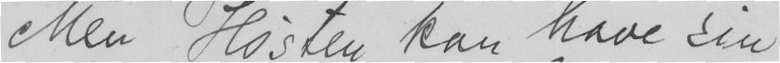Men Høsten kan have sin
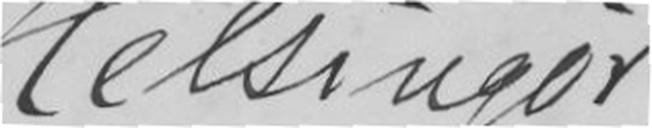Helsingsør.
